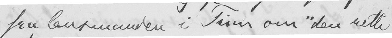fra lensmaaden i Tinn om "den rette
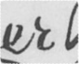er
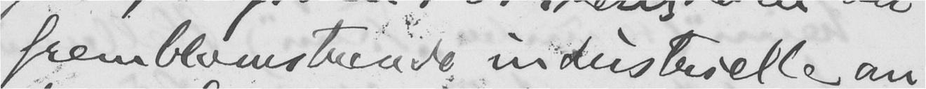fremblomstrende industrielle an-
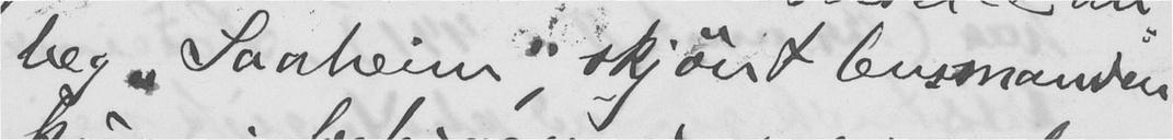læg "Saaheim", skjønt lensmanden
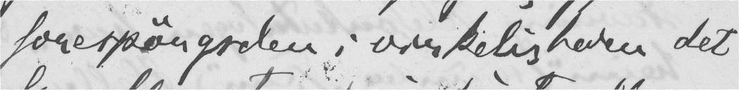forespørgselen i virkeligheden det
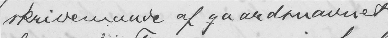skrivemaade af gaardsnavnet
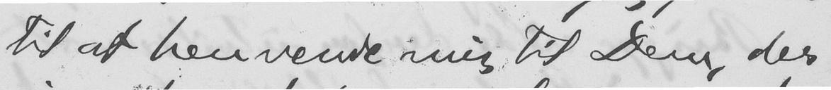til at henvende mig til Dem, der
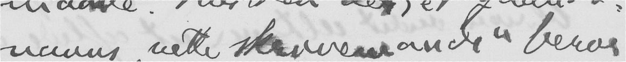navns "rette skrivemaade" beror
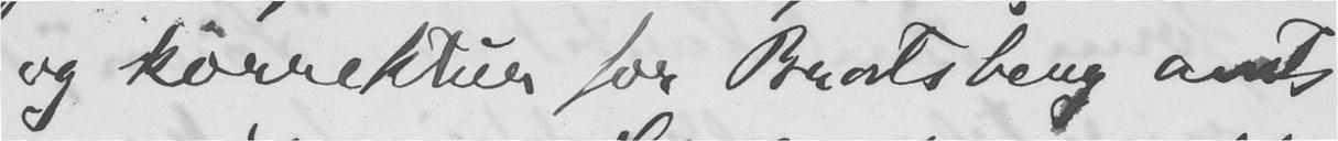og korrektur for Bratsberg amts
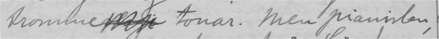trommetonar. Men pianisten,
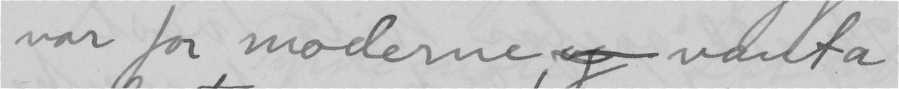var for moderne,  vanta
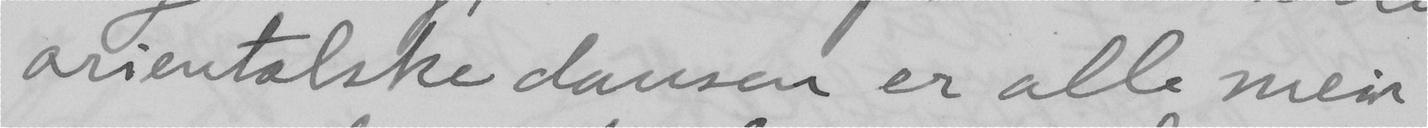orientalske dansen er alle meir
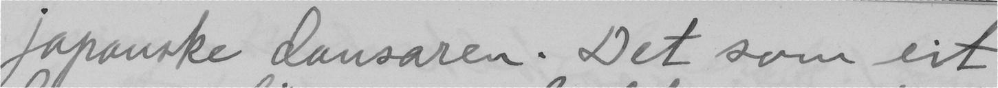japanske dansaren. Det som eit
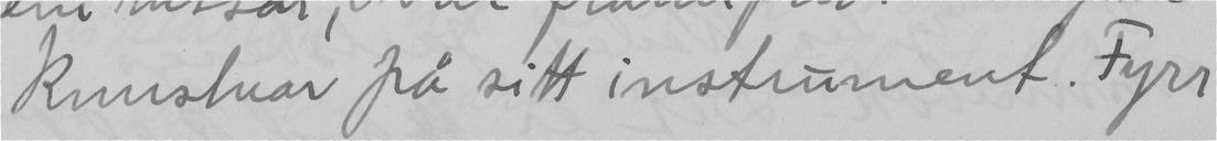kunstnar på sitt instrument. Fyrr
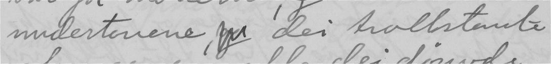undertonene, dei trollstemte
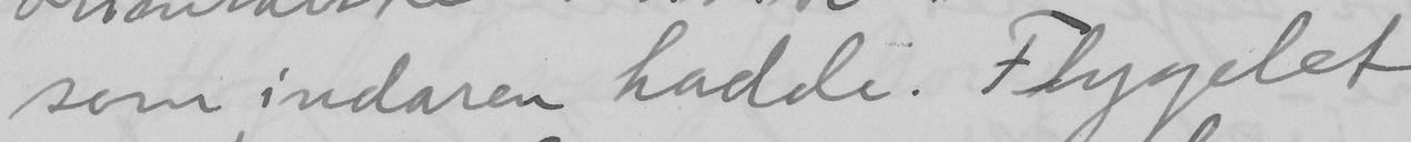som indaren hadde. Flygelet
